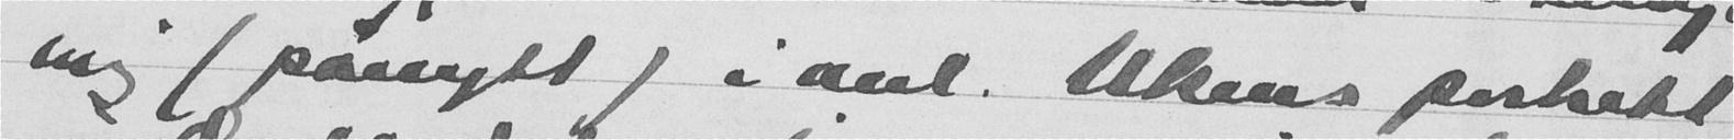mig (pånytt) i anl. Ukens portrett
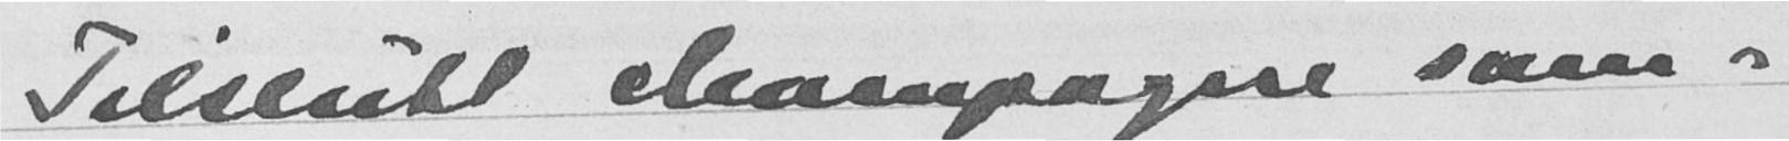Tilslutt champagne sam-
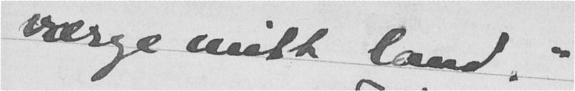værge mitt land."
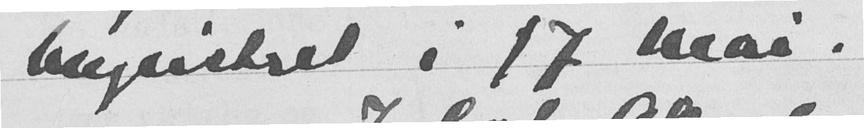begeistret i 17 mai.
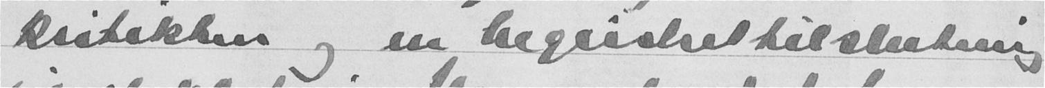kritikken og en begeistret tilslutning
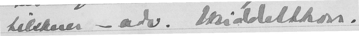tilskuer - adv. Middelthorn.
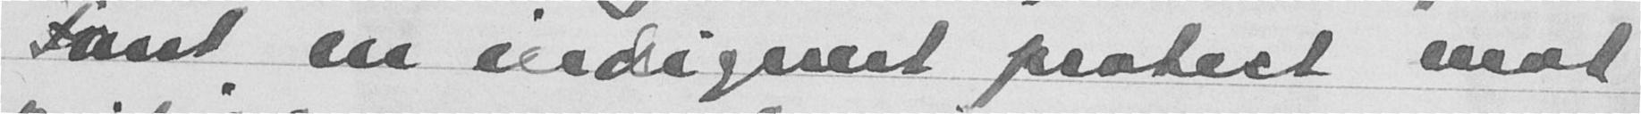Fant en indignert protest mot
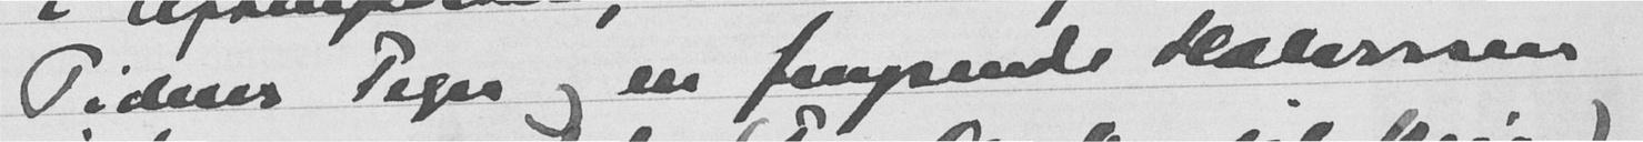Tidens Tegn og en fungerende Halvorsen
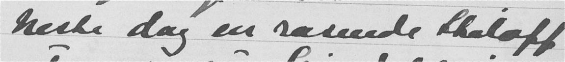Neste dag en rasende Stoloff
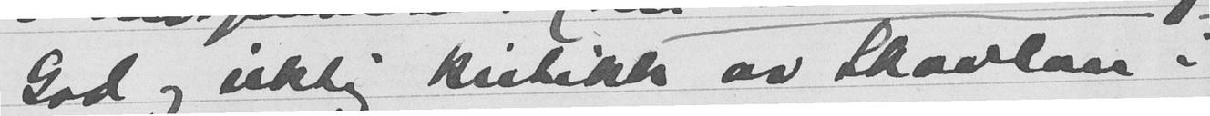God og riktig kritikk av Skavlan i
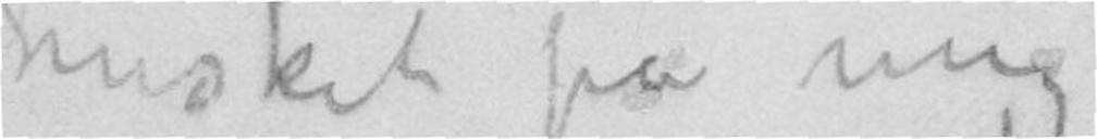husket på mig
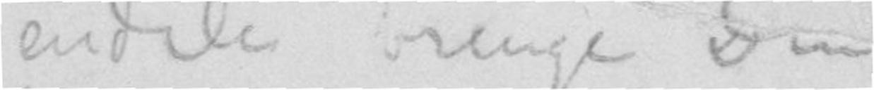endeli bringe Din
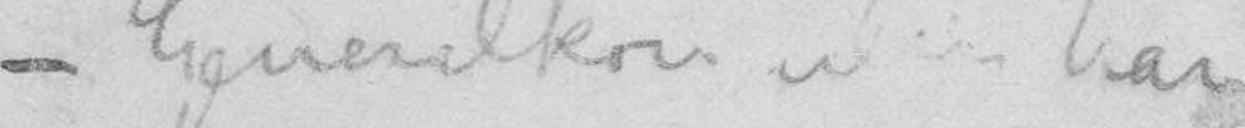– Generalkonsulen har
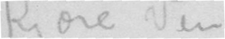Kjære Ven
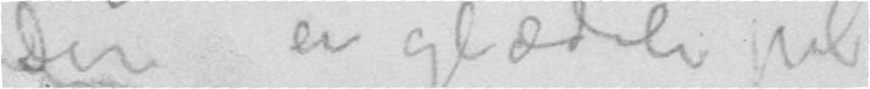Dere en glædeli jul
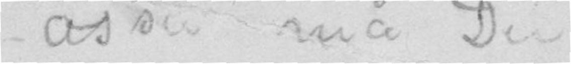– osså må Du
